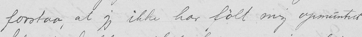forstaa, at jeg ikke har følt mig opmuntret
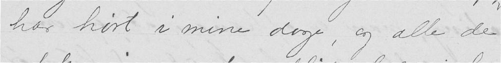har hørt i mine dage, og alle de
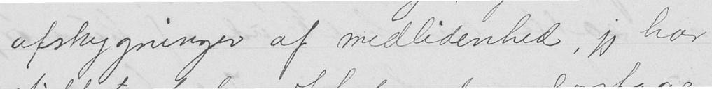afskygninger af medlidenhed, jeg har
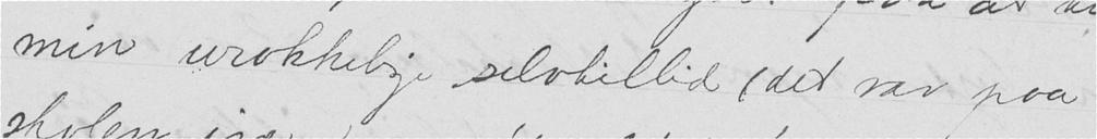min urokkelige selvtillid (det var paa
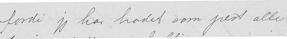fordi jeg har hadet som pest alle
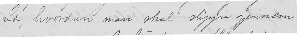ud, hvordan man skal slippe gjennem
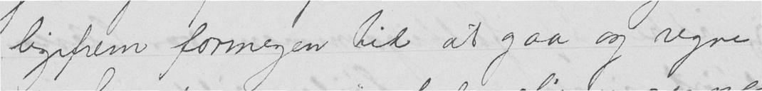ligefrem formegen tid at gaa og regne
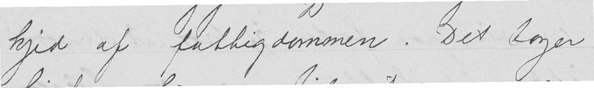kjed af fattigdommen. Det tager
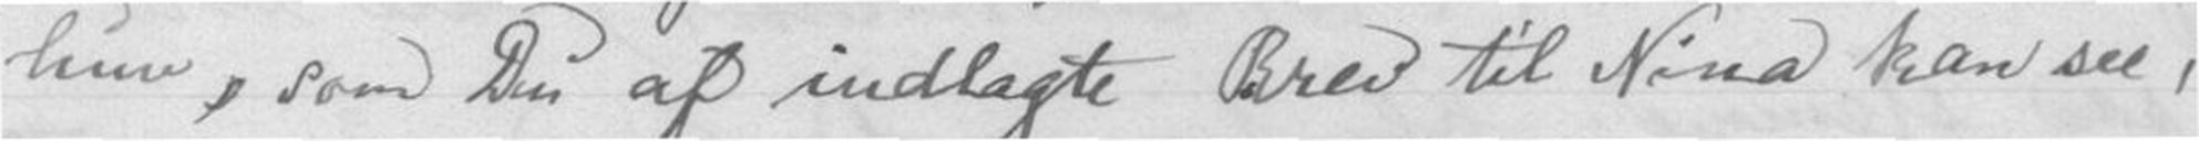hun, som Du af indlagte Brev til Nina kan see,
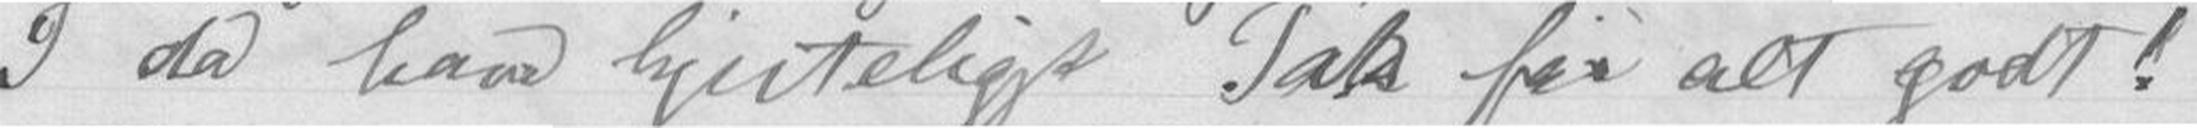I da have hjerteligst Tak for alt godt!
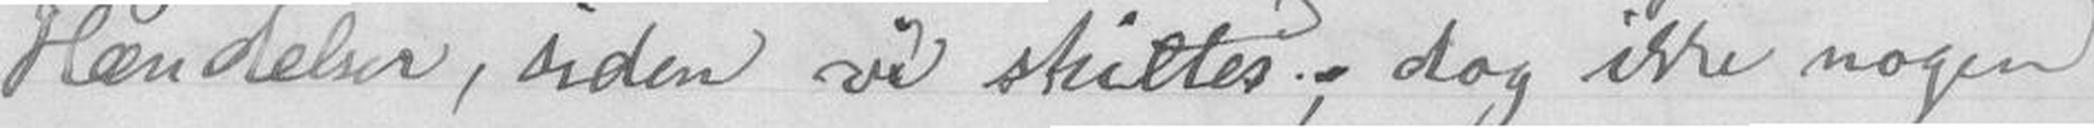Hændelser, siden vi skiltes; dog ikke nogen
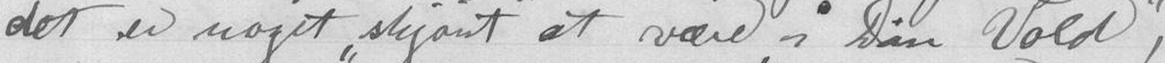det er noget skjønt at være i Din Vold,
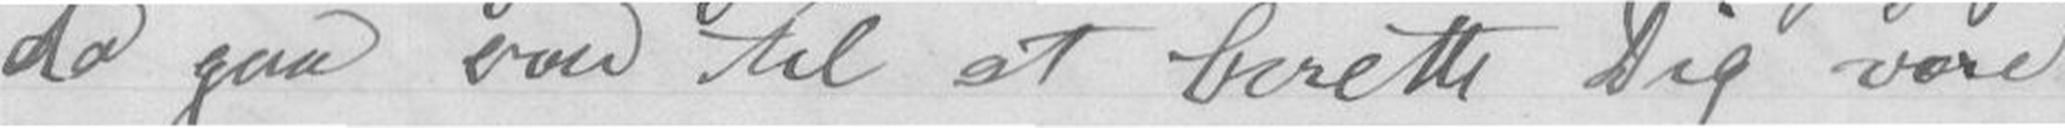da gaa over til at berette Dig vore
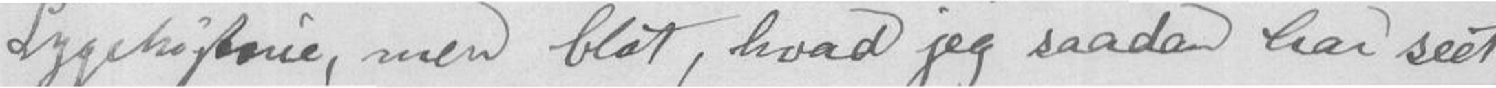Sygehistorie, men blot, hvad jeg saadan har seet
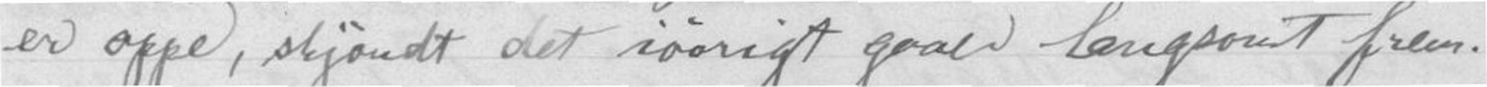er oppe, skjøndt det iøvrigt gaaer langsomt frem.
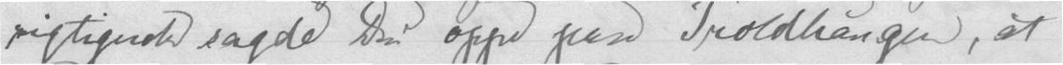rigtignok sagde Du oppe paa Troldhaugen, at
